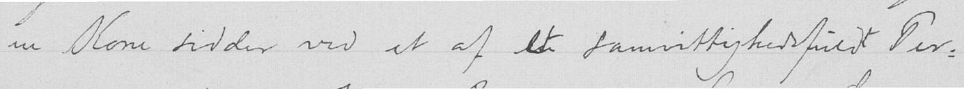en Kone sidder ved et af et samvittighedsfuldt Per-
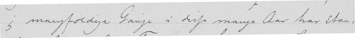jeg mangfoldige Gange i disse mange Aar har stan-
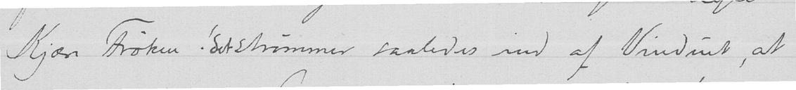Kjære Frøken! det strømmer saaledes ind af Vinduet, at
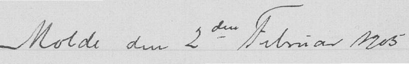Molde den 2den Februar 1905
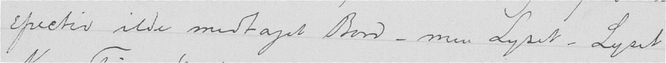spectiv ikke medtaget Bord - men Lyset - Lyset
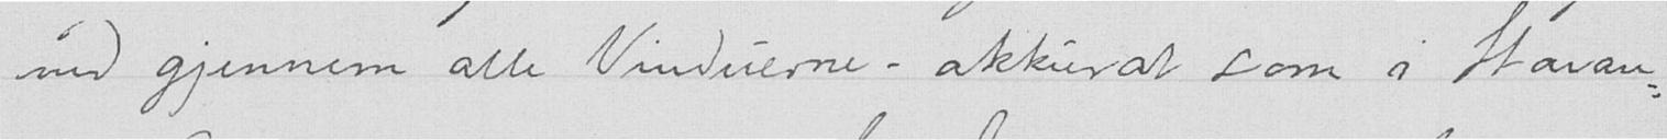ind gjennem alle Vinduerne - akkurat som i Stavan-
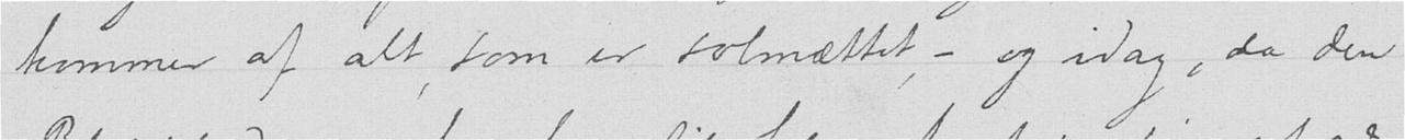kommer af alt, som er solmættet, - og idag, da den
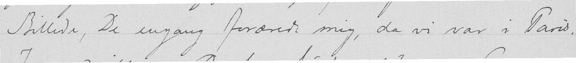Billede, De engang forærede mig, da vi var i Paris.
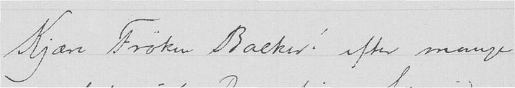Kjære Frøken Backer! efter mange
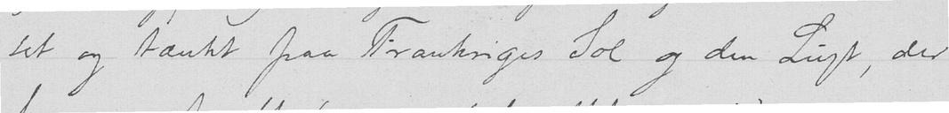set og tænkt paa Frankriges Sol og den Lugt, der
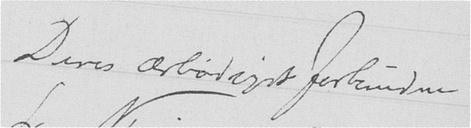Deres ærbødigst forbundne
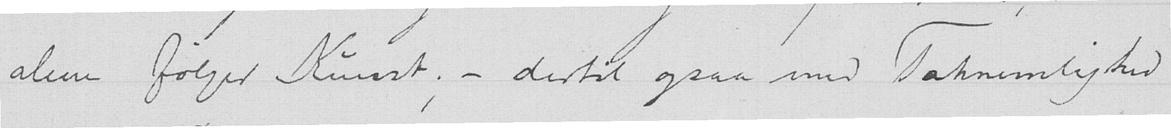alene følger Kunst, - dertil ogsaa med Taknemlighed
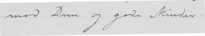mod Dem og gode Minder.
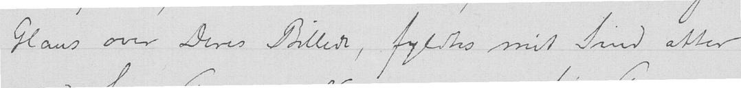Glans over Deres Billede, fyldtes mit Sind atter
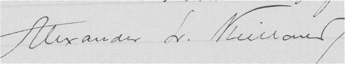Alexander L. Kielland
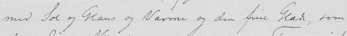med Sol og Glans og Varme og den fine Glæde, som
